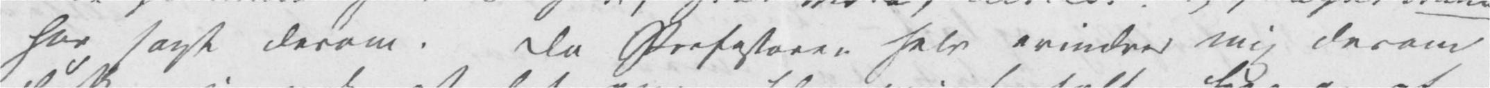har sagt derom. Da Professoren selv erindrer mig derom
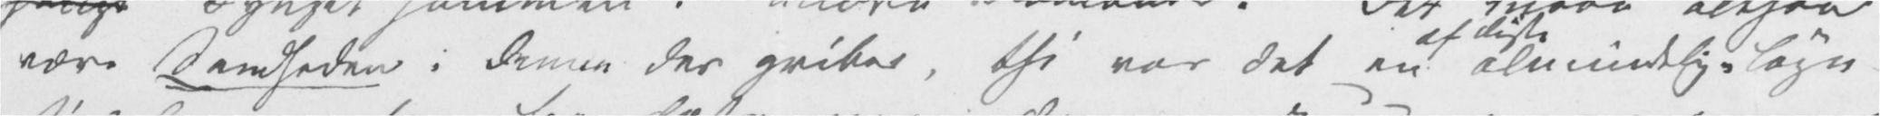skrive i den
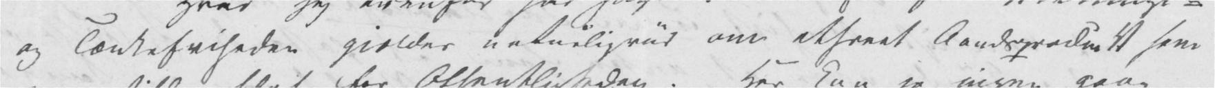og Tænkefriheden gjælder naturligviis om ethvert Aandsproduckt som
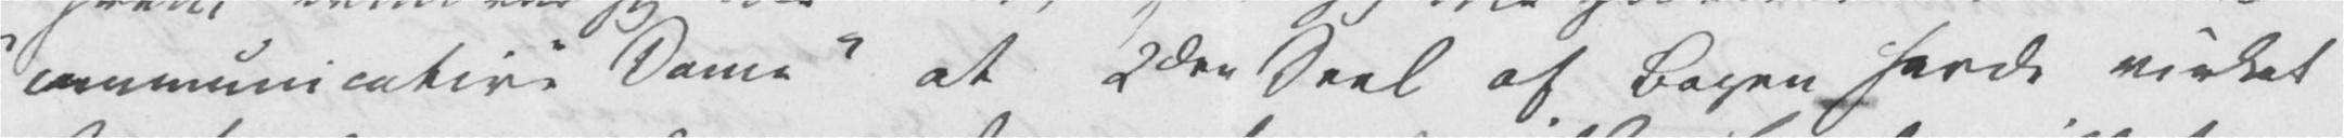«communicative Dame» at 2den Deel af Bogen havde virket
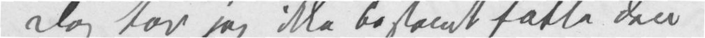Dog tør jeg ikke bestemt fatte den
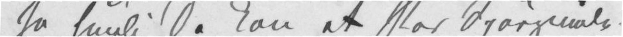så hurtig De kan et Par Spørgsmål.
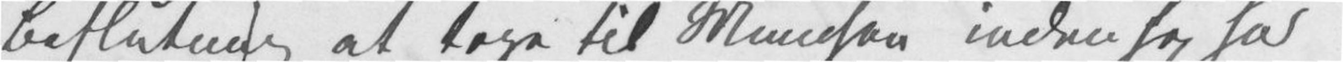Beslutning at tage til München inden jeg har
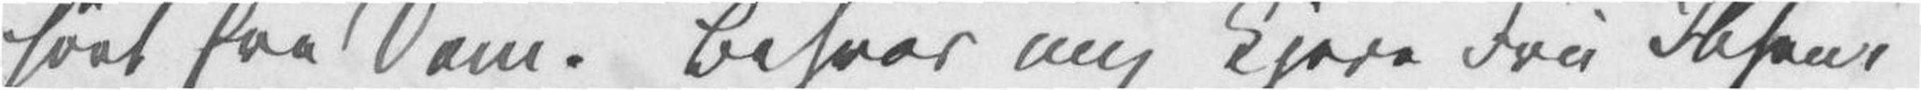hørt fra Dem. Besvar mig kjere Fru Ibsen,
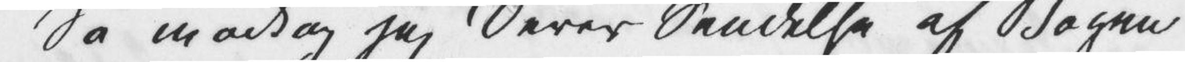Så modtog jeg Deres Sendelse af Bogen
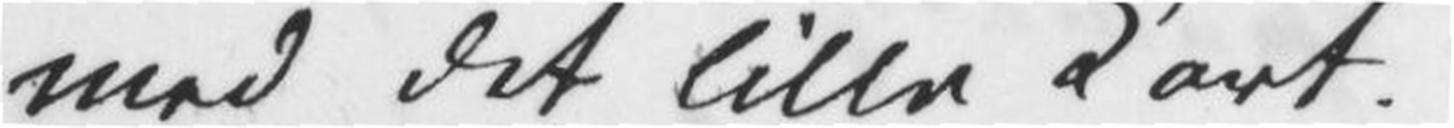med det lille Kort.
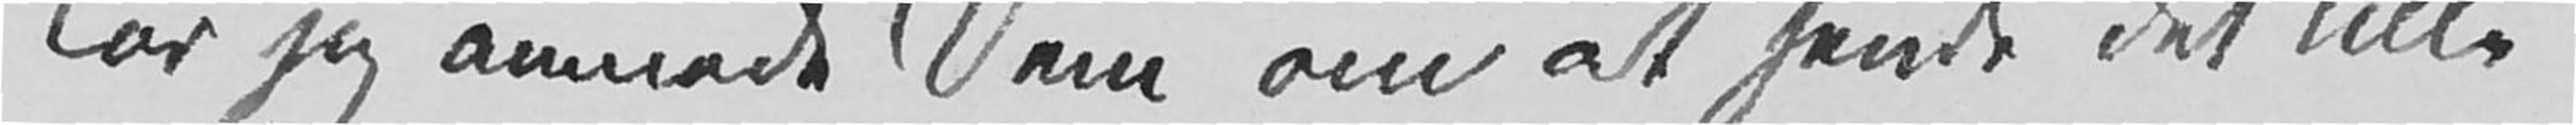Tør jeg anmode Dem om at sende det lille
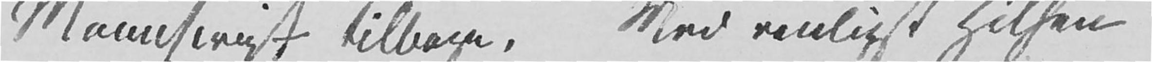Manuscript tilbage. Med venligst Hilsen
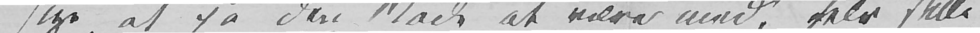sige, at på den Måde at være med, selv stille
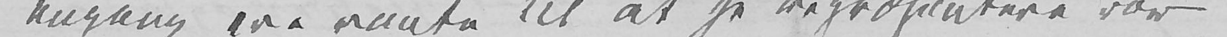engang ere vante til at se repræsentere vor
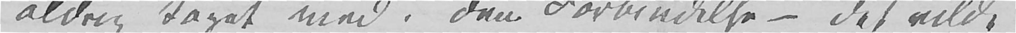aldrig taget med i den Forbindelse – det vilde
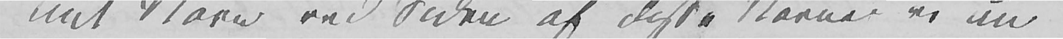mit Navn ved Siden af disse Navne vi nu
